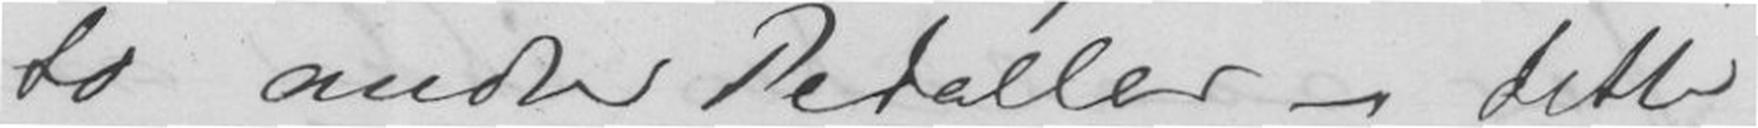to andre Pedaler - dette
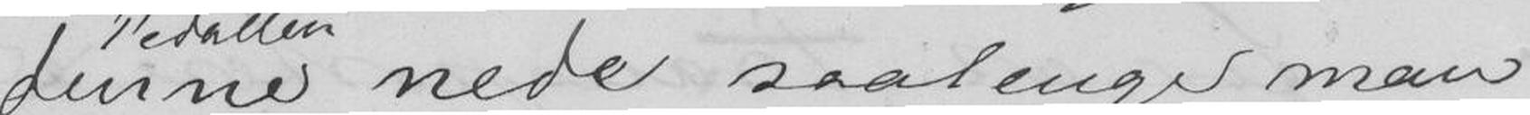denne nede saalenge man
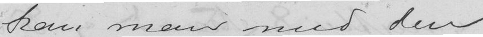kan man med den
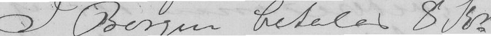I Bergen betales 8 Kr
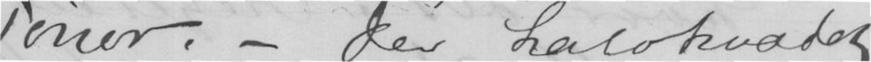Tóner. - Der halvkvædet
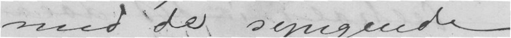med de syngende
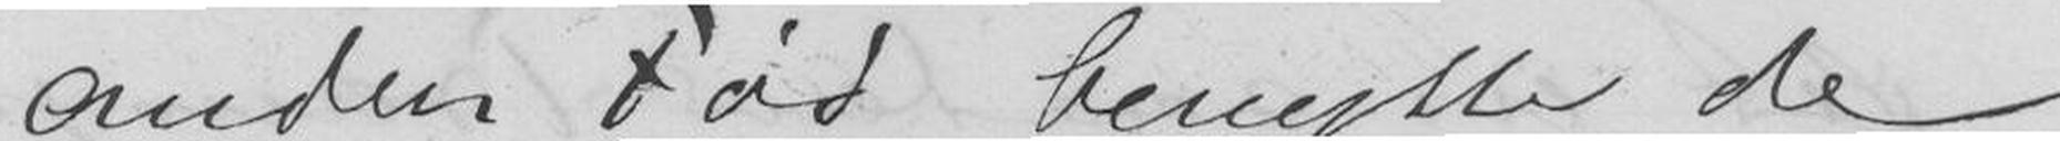anden Fód benytte de
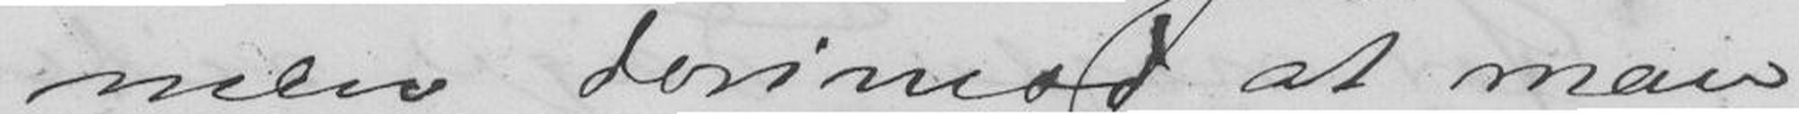men derimod at man
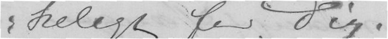keligt for Dig.
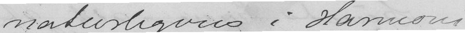naturligviis i Harmoni
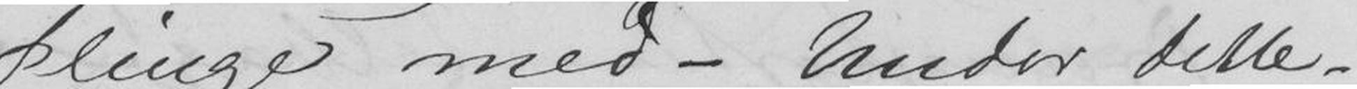klinge med - Under dette
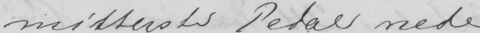mitterste Pedal nede
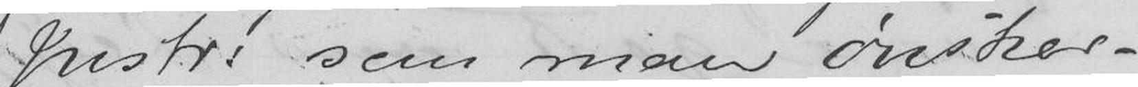Instr: som man ønsker -
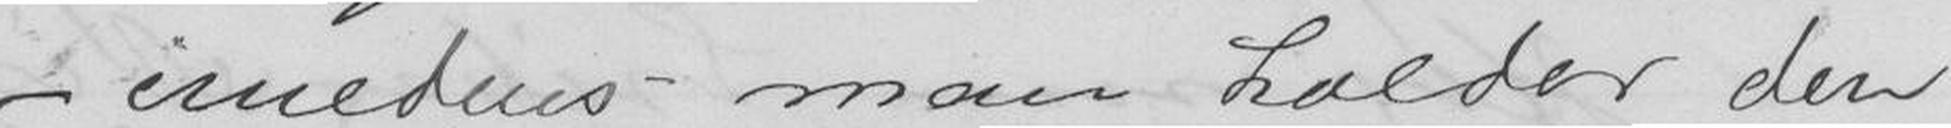- imedens man holder den
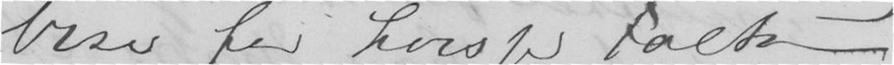vise for hvisse Folk
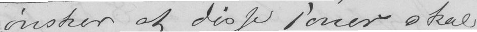ønsker at disse Toner skal
